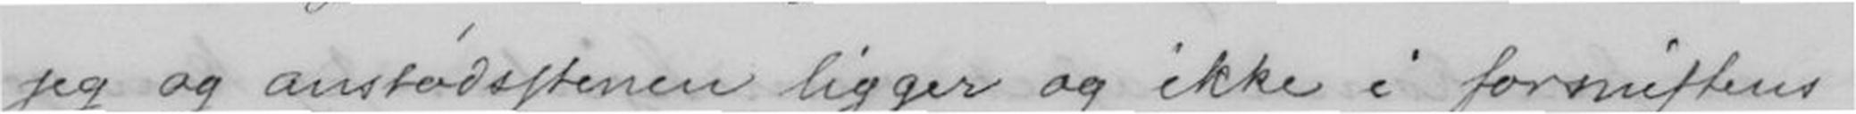jeg og anstødsstenen ligger og ikke i fornuftens
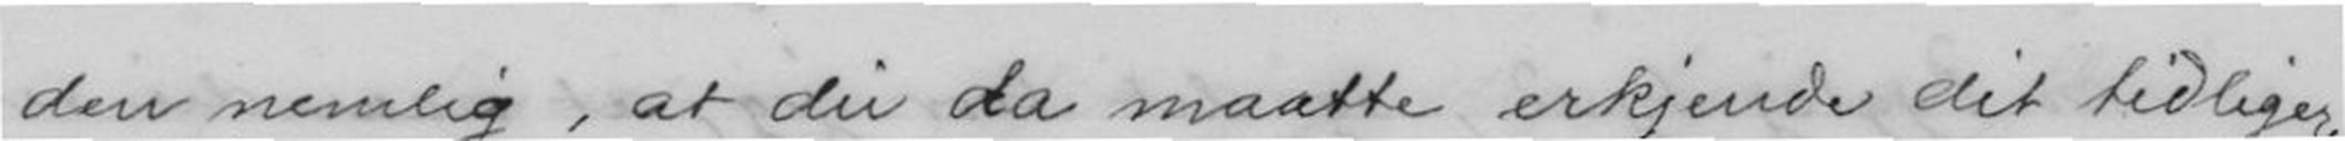den nemlig, at du da maatte erkjende det tidligere
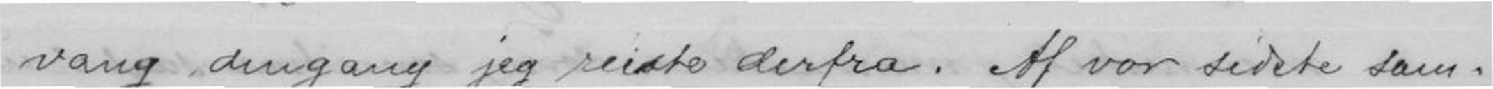vang dengang jeg reiste derfra. Af vor sidste sam
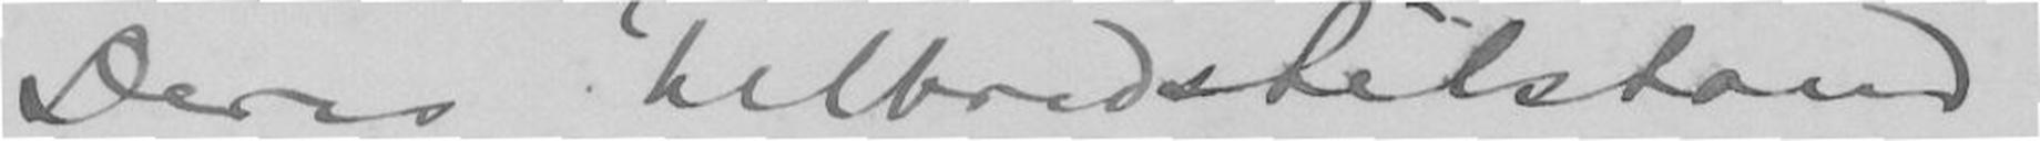Deres helbredstilstand
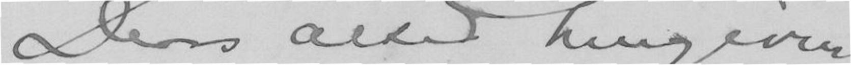Deres altid hengivne
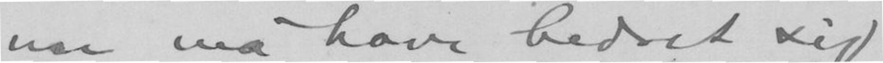nu må have bedret sig
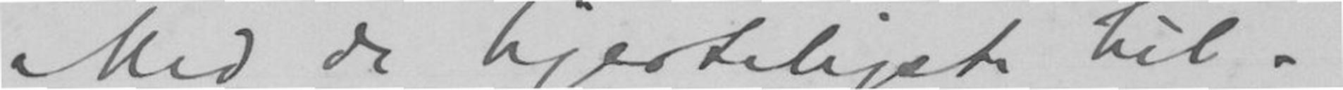Med de hjerteliste hil-
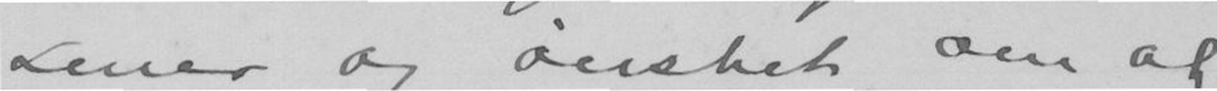sener og ønsket om at
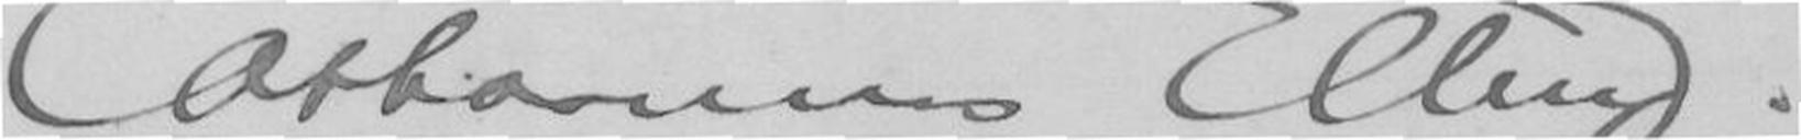Catharinus Elling.
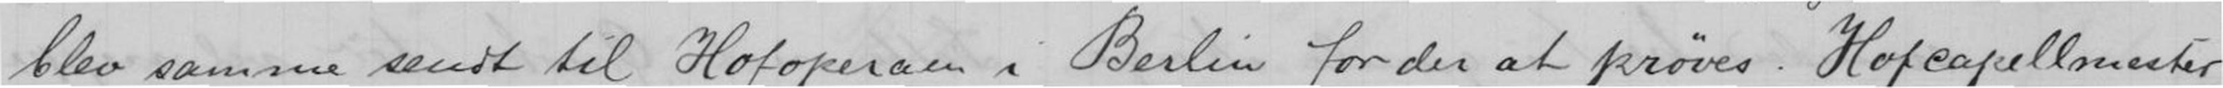blev samme sendt til Hofoperaen i Berlin for at prøves. Hofcapellmester
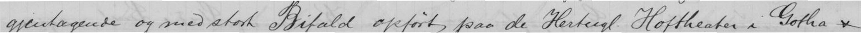gjentagende og med stort Bifald opført paa de Hertugl Hoftheater i Gotha &
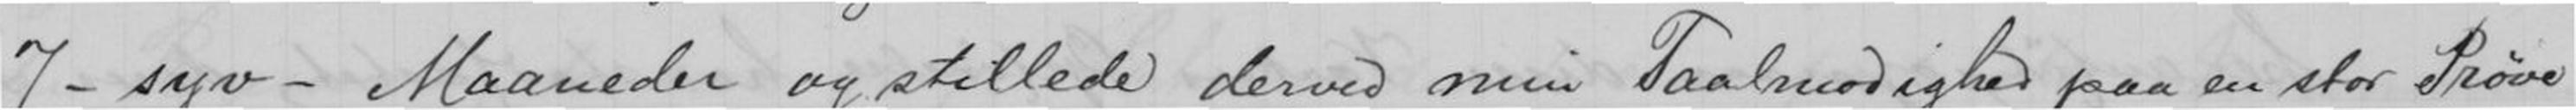7-syv- Maaneder og stillede derved min Taalmodighed paa en stor Prøve
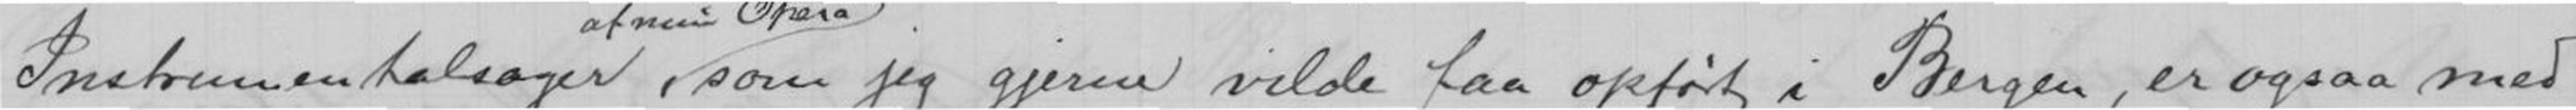Instrumentalsager som jeg gjerne vilde faa opført i Bergen, er ogsaa med
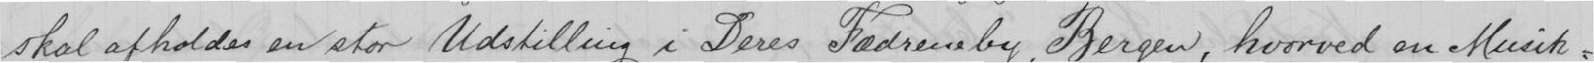skal afholdes en stor Udstilling i Deres Fædreneby, Bergen, hvorved en Musik-
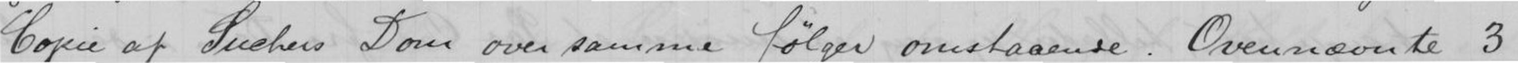Copi af Suchers Dom over samme følger omstaaende. Ovennævnte 3
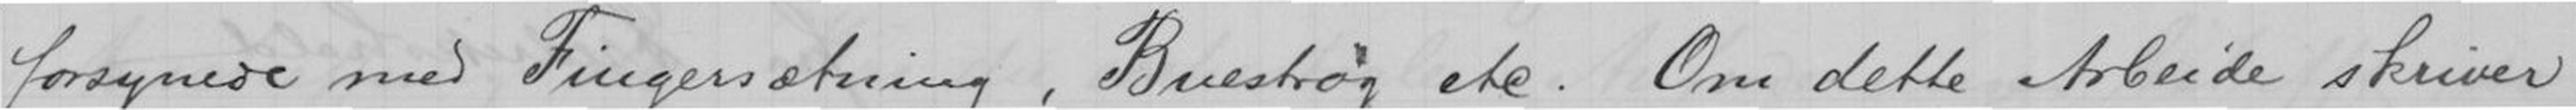fornyede med Fingersætning, Buestrøg etc. Om dette Arbeide skriver
